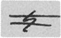#
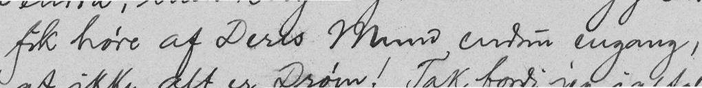fik høre af Deres Mund endnu engang,
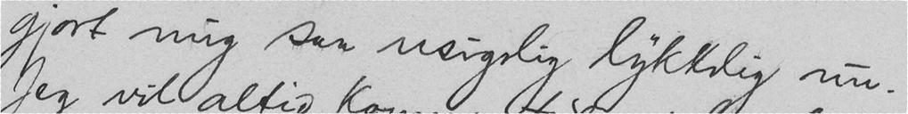gjort mig saa usigelig lykkelig nu.
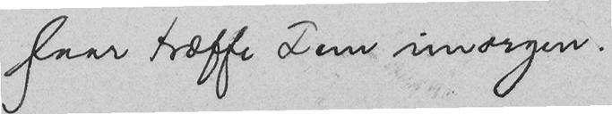faar træffe Dem imorgen.
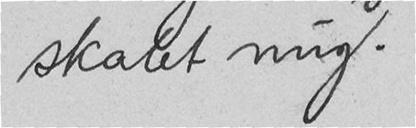skabt mig.
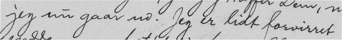jeg nu gaar ud. Jeg er lidt forvirret
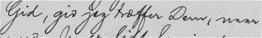Gid, gid jeg træffer Dem, naar
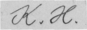K. H.
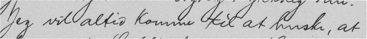Jeg vil altid komme til at huske, at
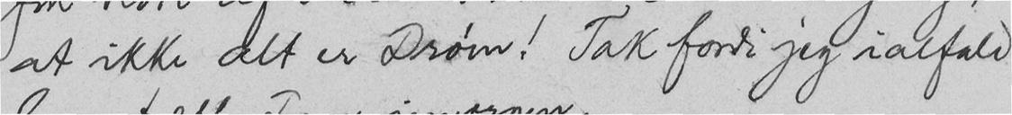at ikke alt er Drøm! Tak fordi jeg ialfald
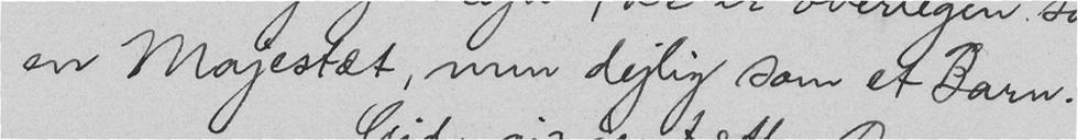en Majestæt, men dejlig som et Barn.
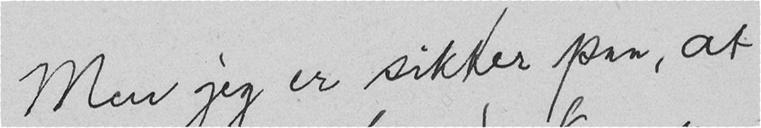Men jeg er sikker paa, at
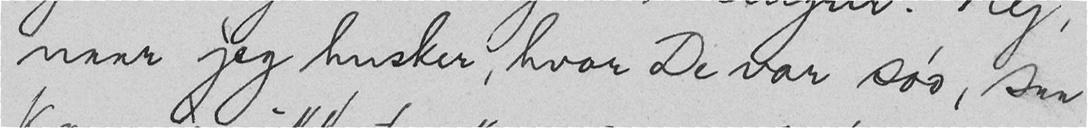naar jeg husker, hvor De var sød, saa
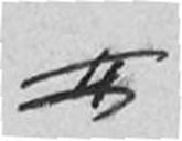#
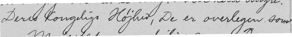Deres Kongelige Højhed, De er overlegen som
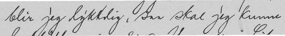blir jeg lykkelig, saa skal jeg kunne
